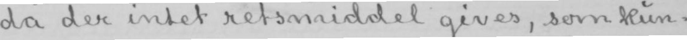da der intet retsmiddel gives, som kun-
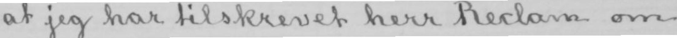at jeg har tilskrevet herr Reclam om
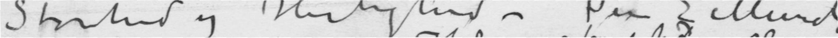Storhed og Herlighed – Din E Munch
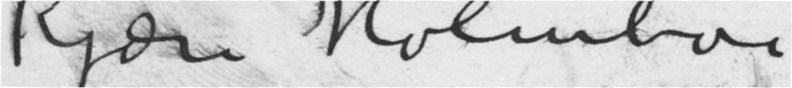Kjære Holmboe
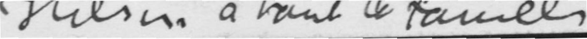Hilsen a toute & Famille
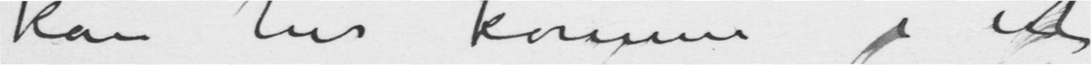kan her komme i et
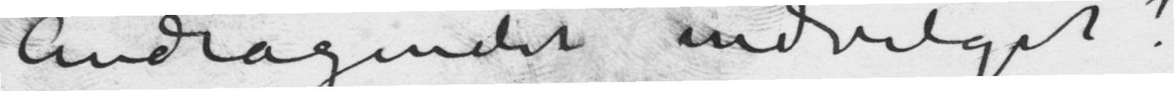Andragendet indvilget?
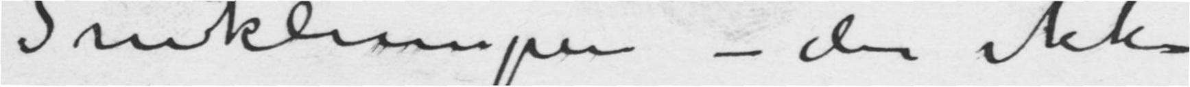Sneklumpen – der ikke
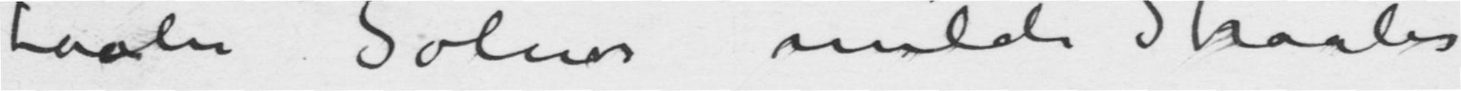taaler Solens milde Straaler
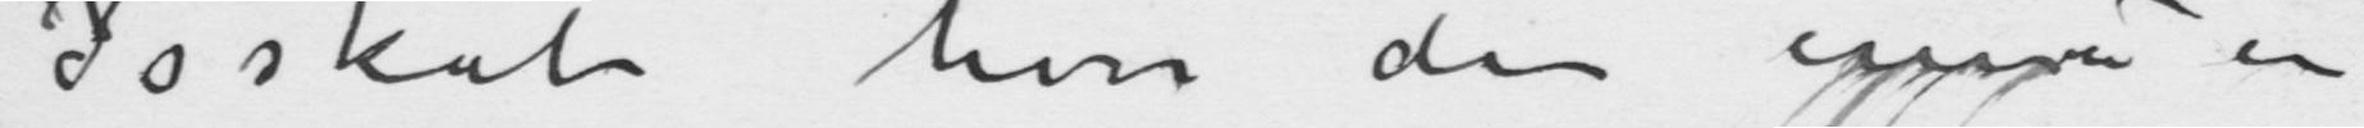Isskab hvor den ennu en
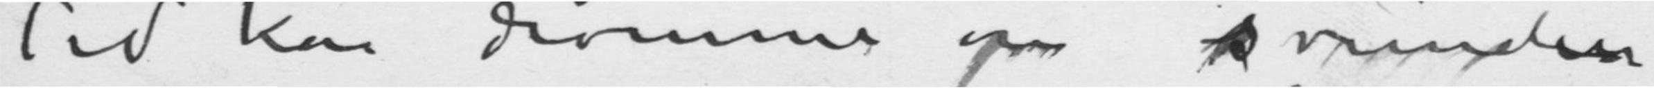Tid kan drømme om svunden
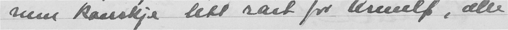men kanskje litt rart for Arnulf, alle
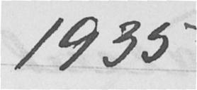1935
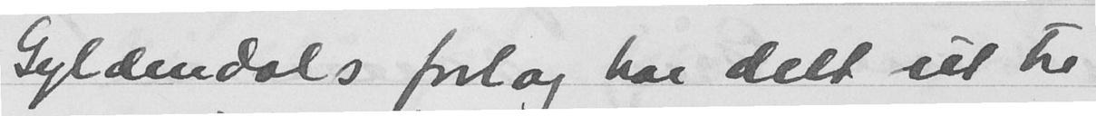Gyldendals forlag har delt ut tre
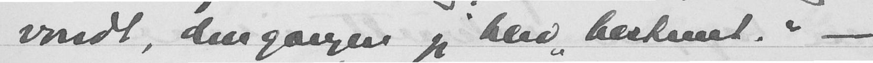vondt, dengangen jeg blev "bestemt." -
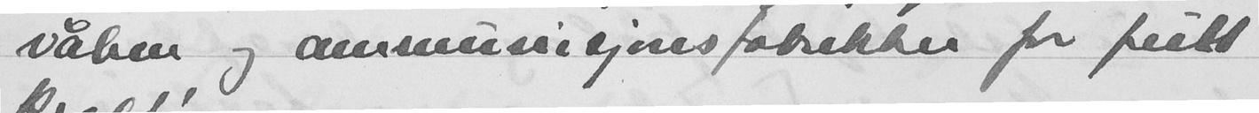våben og ammunisjonsfabrikker for feitt
